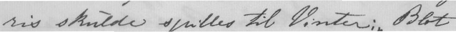ris skulde spilles til Vinter; Blot
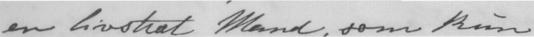en Livstræt Mand, som kun
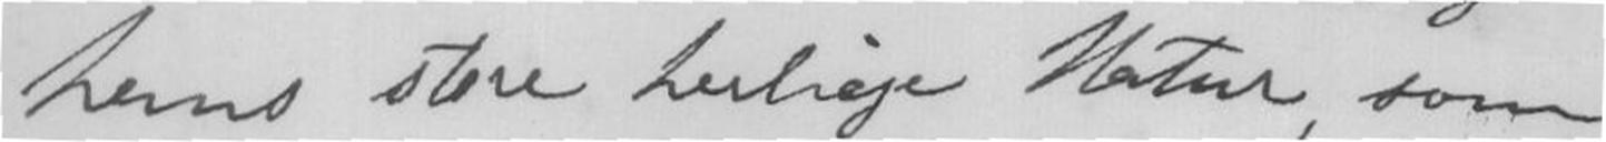hans store herlige Natur, som
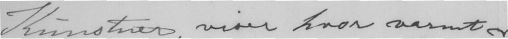Kunstner, viser hvor varmt
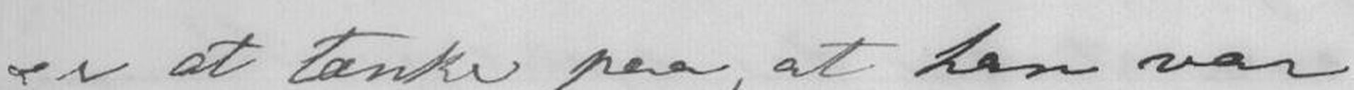er at tænke paa, at han var
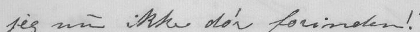jeg nu ikke dør forinden!
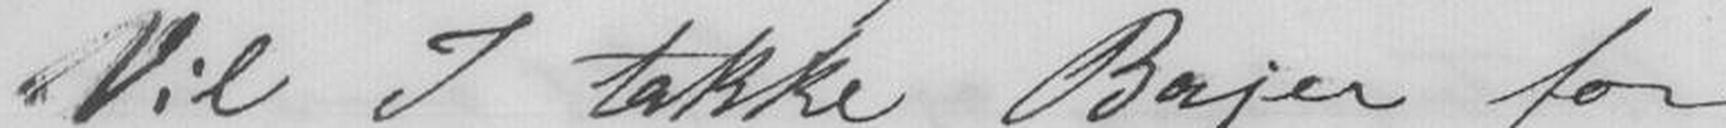Vil I takke Bajer for
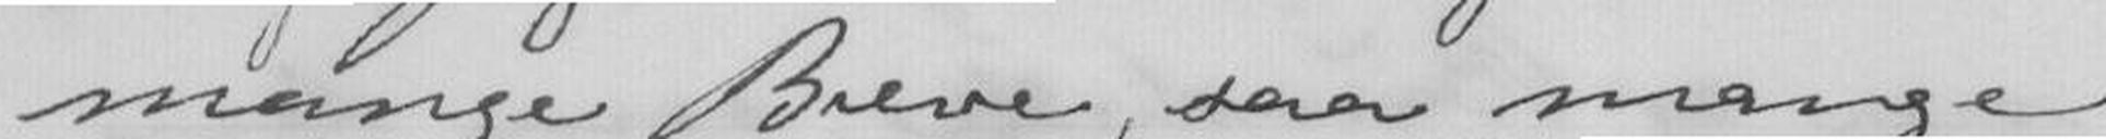mange Breve, saa mange
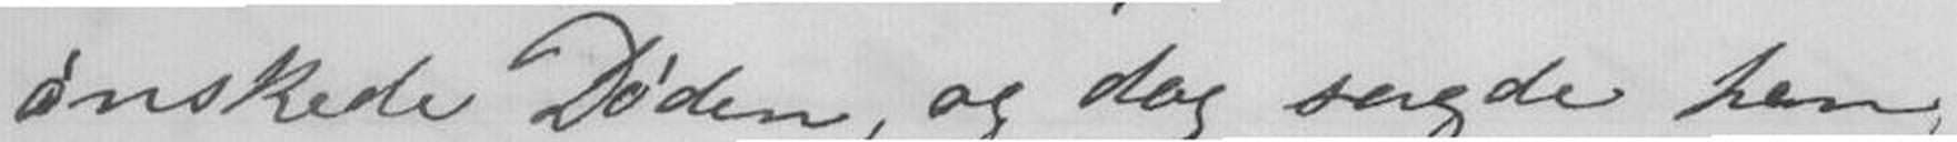ønskede Døden, og dog sagde han,
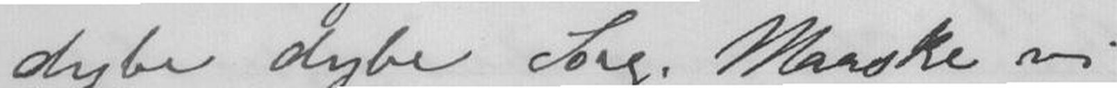dybe dybe Sorg. Maaske vi
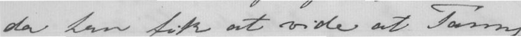da han fik at vide at Tamy-
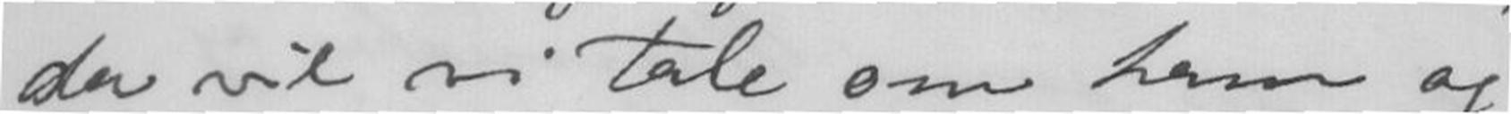da vil vi tale om ham og
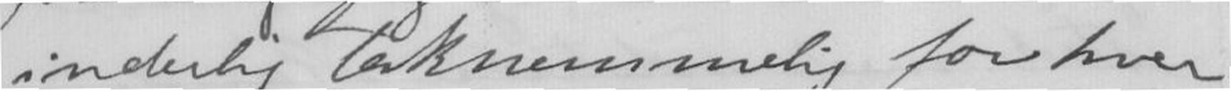inderlig taknemmlige for hver
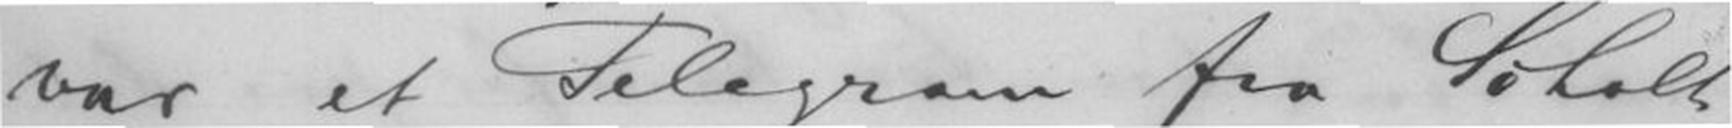var et Telegram fra Søholt
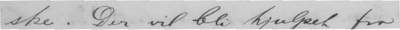ske. Der vil bli hjulpet fra
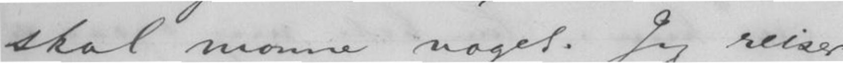skal monne noget. Jeg reiser
